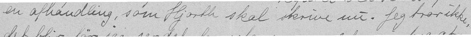en afhandling, som Hjorth skal skrive nu. Jeg tror ikke
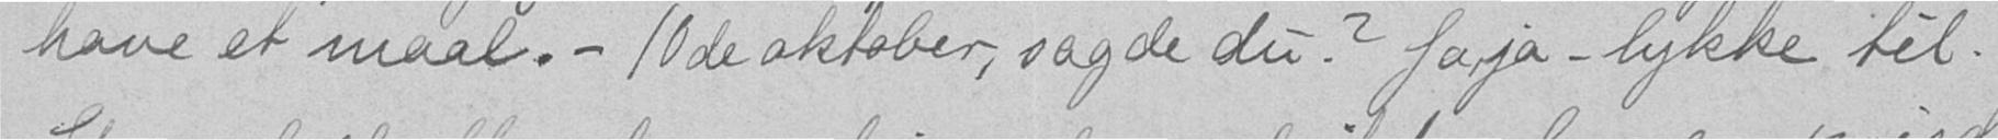have et maal.- 10de oktober, sagde du? Jaja - lykke til!
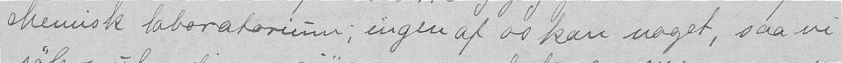chemisk laboratorium; ingen af os kan noget, saa vi
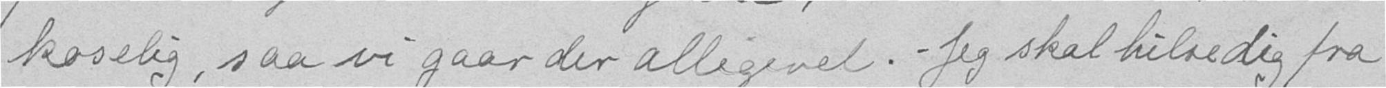koselig, saa vi gaar der alligevel. - Jeg skal hilse dig fra
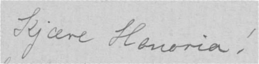Kjære Honoria!
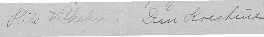Hils Vilhelm! Din Kristine.
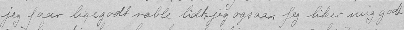jeg faar ligegodt rable lidt jeg ogsaa. Jeg liker mig godt
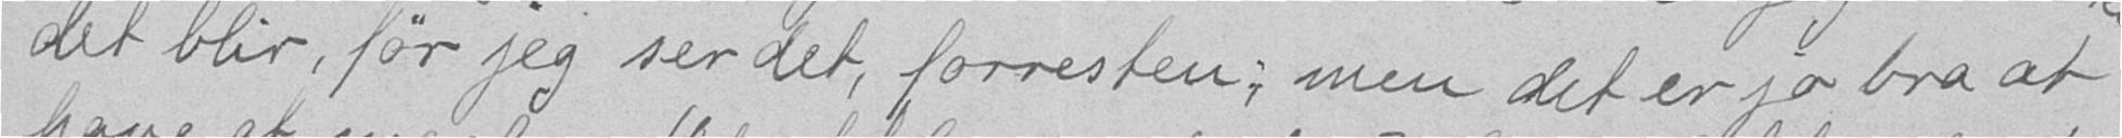det blir, før jeg ser det, forresten; men det er jo bra at
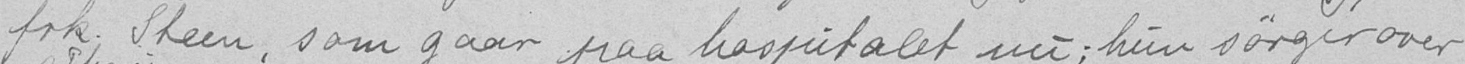frk. Steen, som gaar paa hospitalet nu; hun sørger over
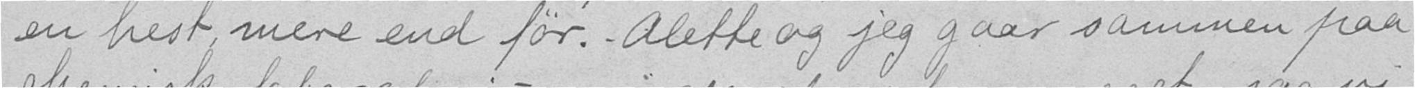en hest, mere end før. - Alette og jeg gaar sammen paa
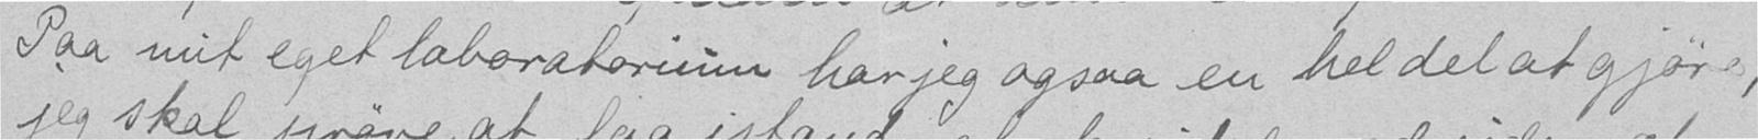Paa mit eget laboratorium har jeg ogsaa en hel del at gjøre,
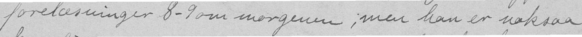forelæsninger 8-9 om morgenene; men han er noksaa
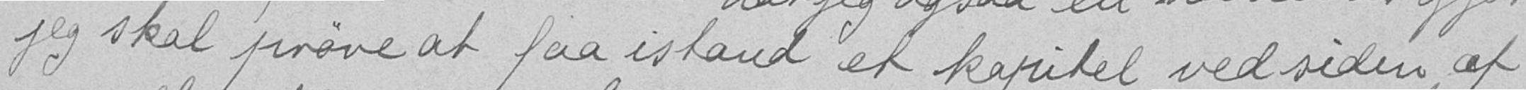jeg skal prøve at faa istand et kapitel ved siden af
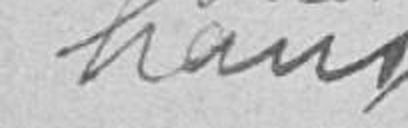hans
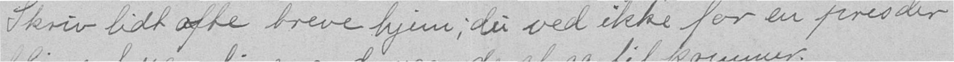Skriv lidt ofte breve hjem; du ved ikke for en pris der
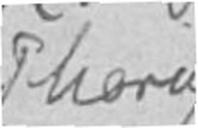Thorups
